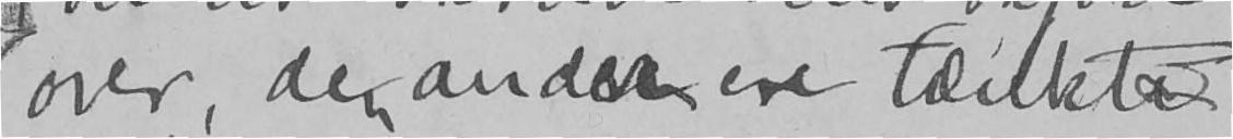over, den anden ere tænkte
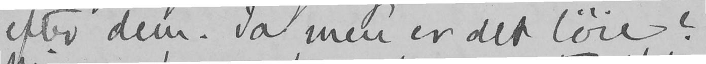efter dem. Ja men er det løie?
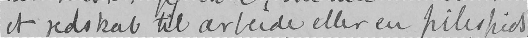et redskab til arbeide eller en pilespids.
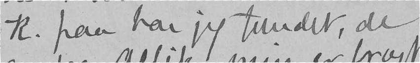K. paa har jeg fundet, de
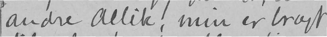andre Allik, min er brugt
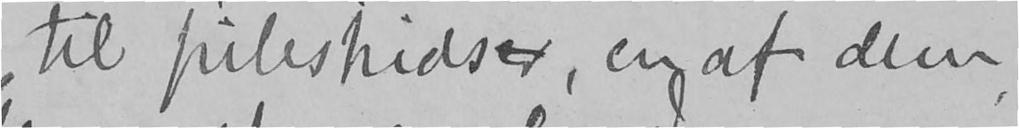til pilespidser, en af dem,
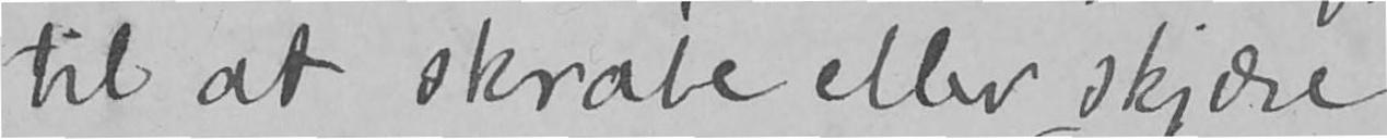til at skrabe eller skjære
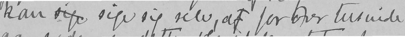kan sige sige sig selv, at for over tusinde
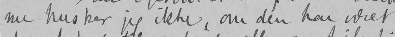nu husker jeg ikke, om den har været
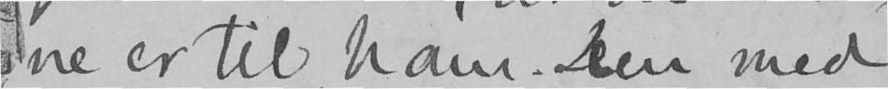ne er til ham. Den med
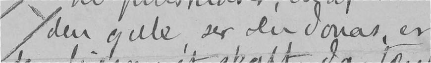den gule, ser Du Jonas, er
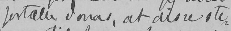fortælle Jonas, at disse ste-
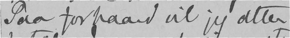Paa forhaand vil jeg atter
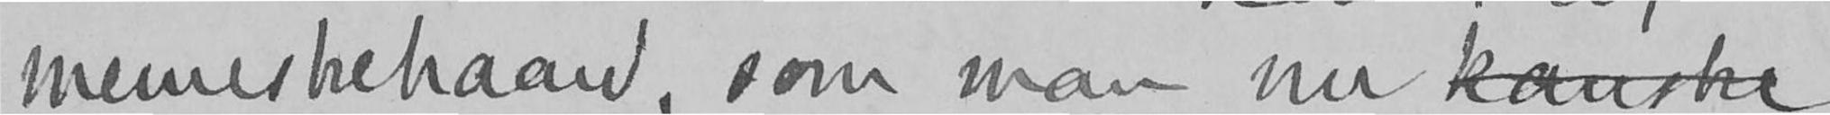menneskehaand, som man nu kanske
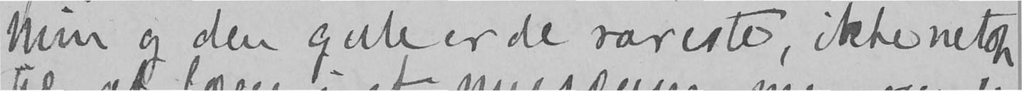Min og den gule er de rareste, ikke netop
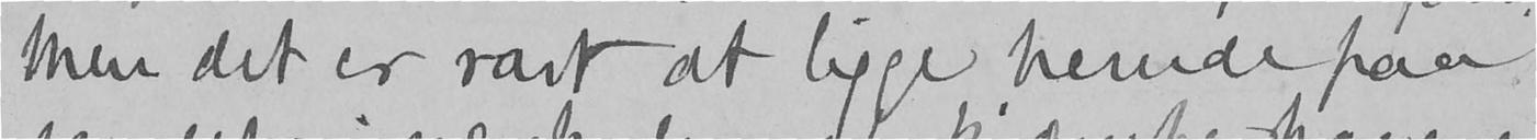Men det er rart at ligge herude paa
